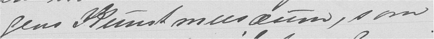gens Kunstmusæum, som
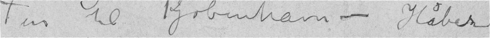Tur til Kjøbenhavn – Håber
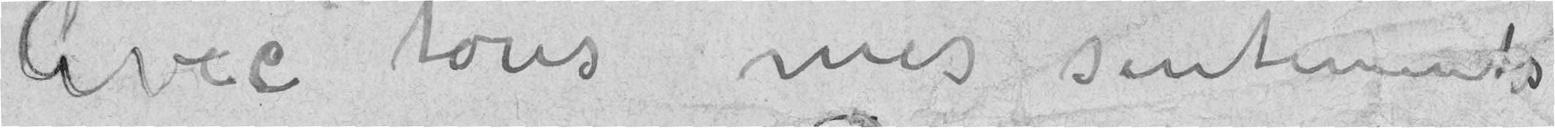Avec tous mes sentiments
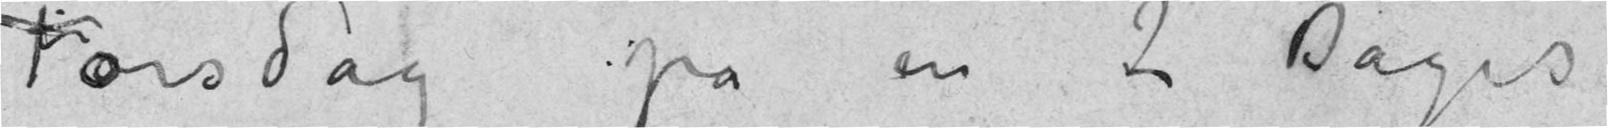Torsdag på en 2 Dages
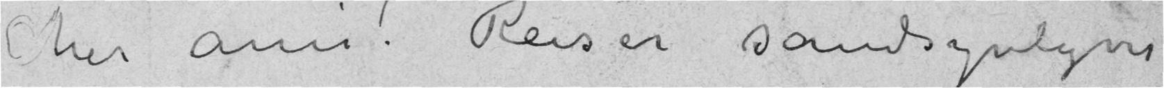Cher ami! Reiser sandsynligvis
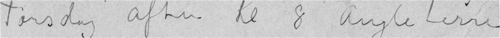Torsdag aften kl 8 Angleterre
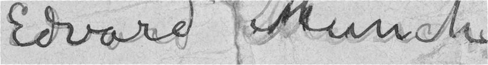Edvard Munch
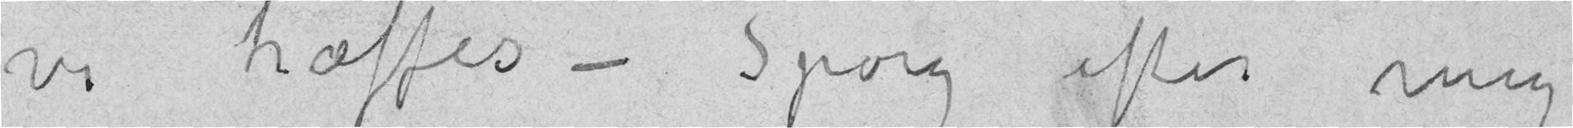vi træffes – Spørg efter mig
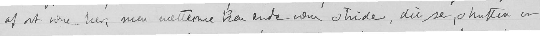af at være her, men nætterne kan enda være stride, du ser, skriften er
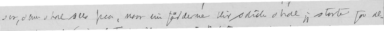æer, som skal sees paa, naar nu fødderne blir solide skal jeg starte for al
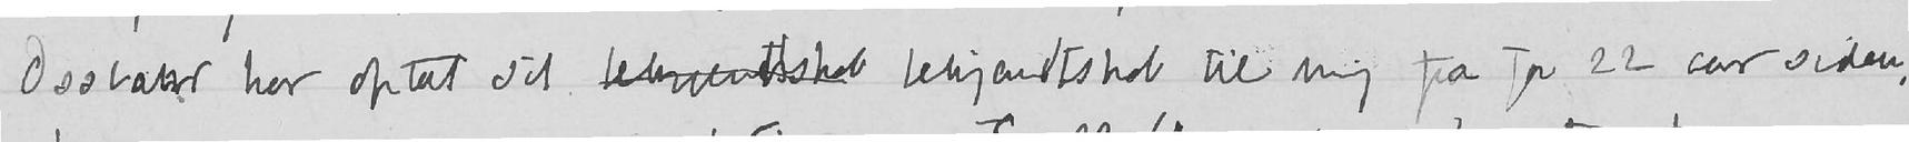Ossbahr har optat sit bekjentsskab bekjendtskab til mig fra for 22 aar siden,
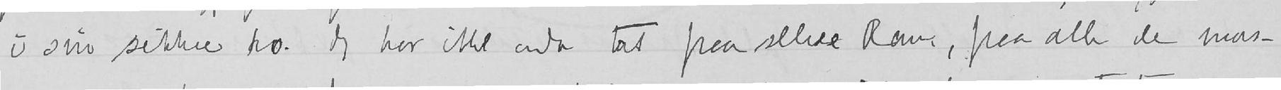i sin sikkre ro. Jeg har ikke enda tat paa selve Rom, paa alle de mus-
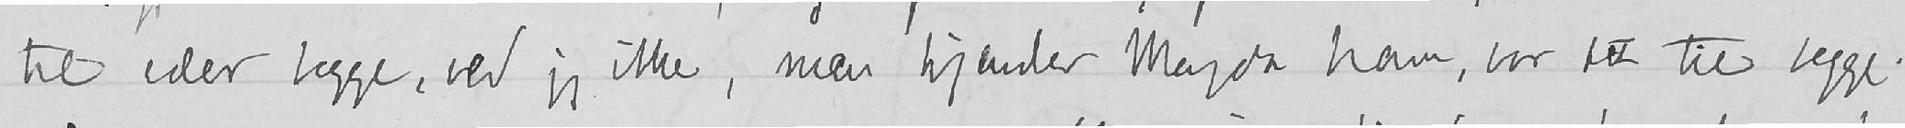til eder begge, ved jeg ikke, men kjender Magda ham, var det til begge.
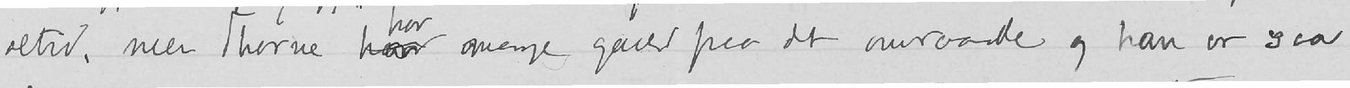altid, men Thorne han mange gaver paa det omraade og han er saa
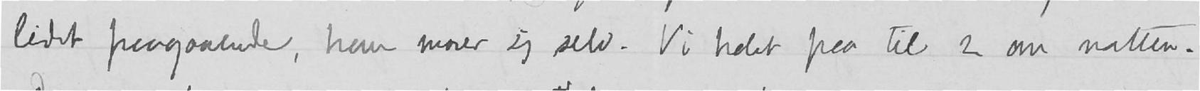lidet paagaaende, han morer sig selv. Vi holdt paa til 2 om natten.
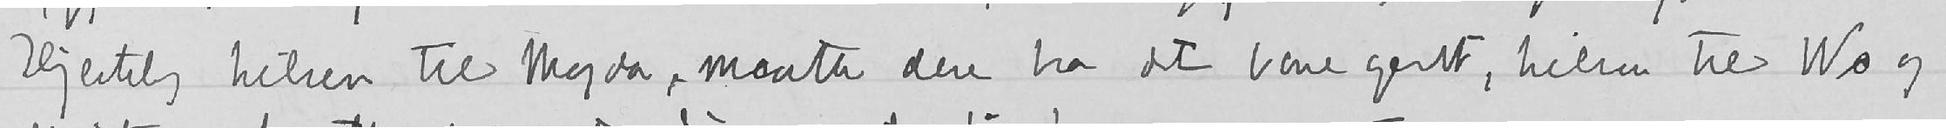Hjertelig hilsen til Magda, maatte dere ha det bare godt, hilsen til Ws og
